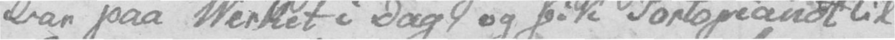var paa Verket i Dag, og fik Portopianot til
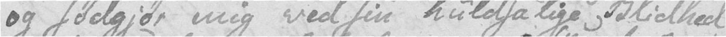og sødgjør mig ved sin huldsalige Blidhed
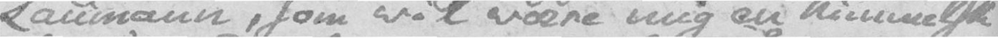Laumann, som vil være mig en himmelsk
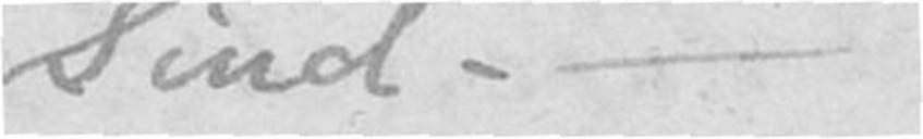Sind. –
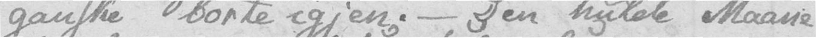ganske borte igjen. – Den hulde Maane
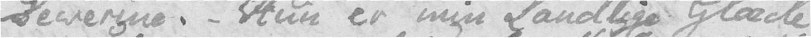Severine. – Hun er min Landlige Glæde
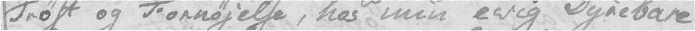Trøst og Fornøjelse, hos min evig Dyrebare
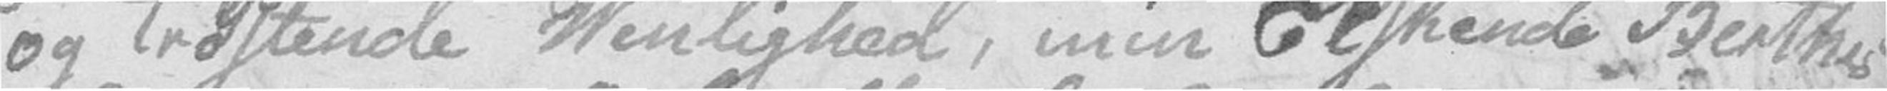og trøstende Venlighed, min Elskende Berthas
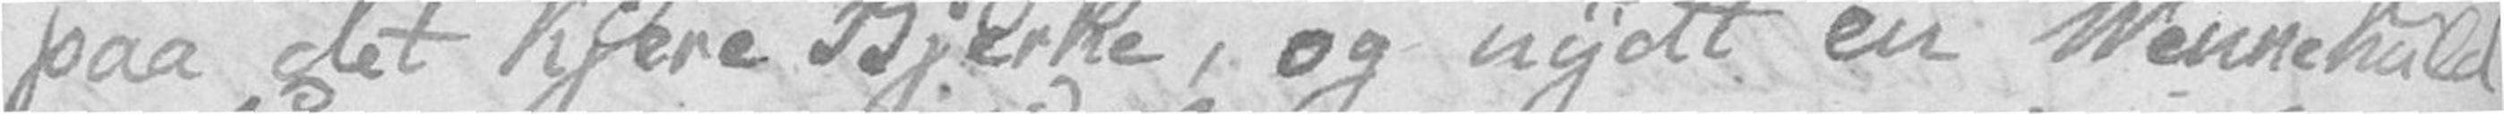paa det kjere Bjerke, og nydt en Vennehuld
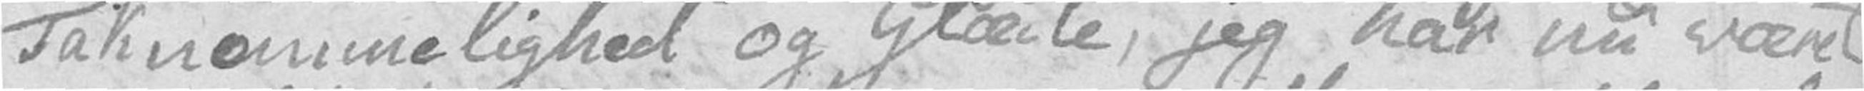Taknemmelighed og Glæde, jeg har nu været
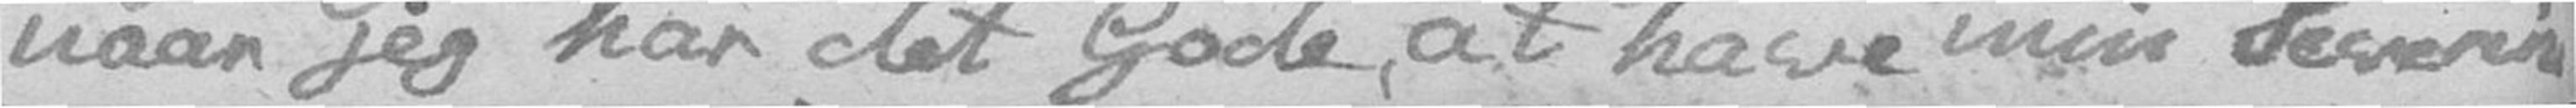naar jeg har det Gode, at have min Severine
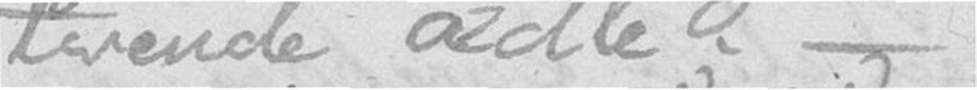tvende ædle. –
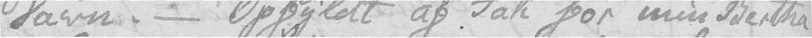Savn. – Opfyldt af Tak for min Bertha
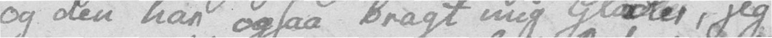og den har ogsaa bragt mig Glæder, jeg
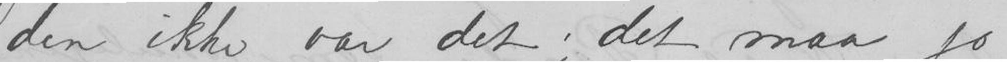den ikke var det; det maa jo
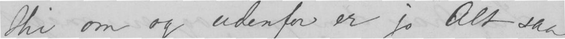thi om og udenfra er jo Alt saa
